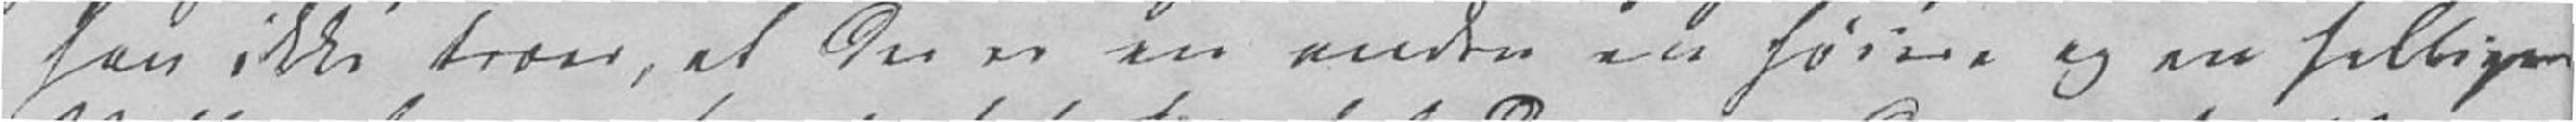han ikke troer, at den er en anden en høiere og en helligere
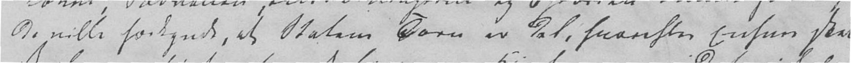de ville forkynde, at Statens Tarv er det, hvorefter Enhver skal
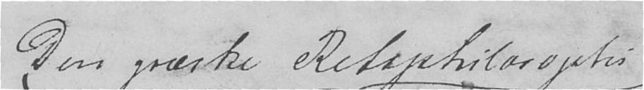Den græske Retsphilosophi
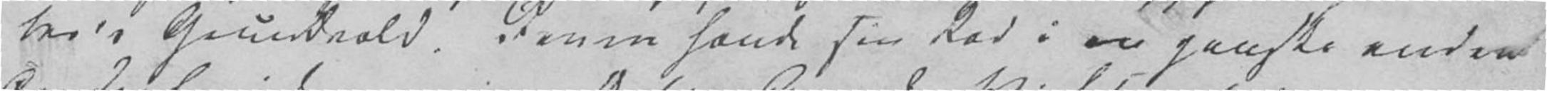tes's Grundvold. Denne havde sin Rod i en ganske anden
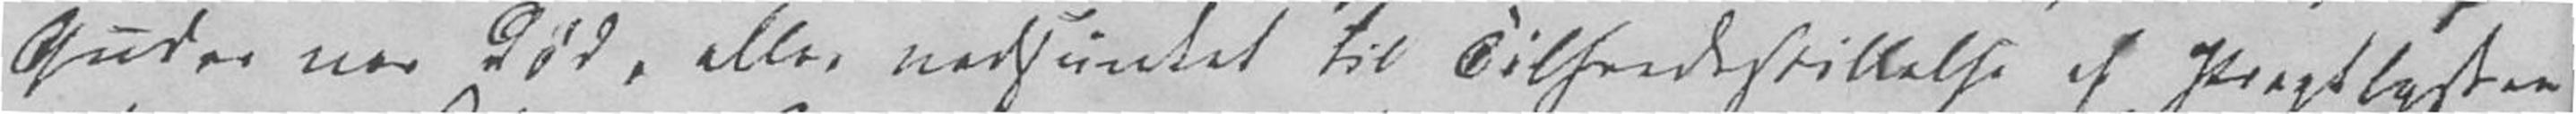Guder var død, eller nedsunket til Tilfredsstillelse af Pragtlysten
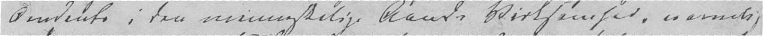Tendents i den menneskelige Aands Virksomhed, nemlig
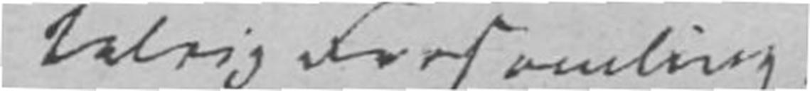talrig Forsamling
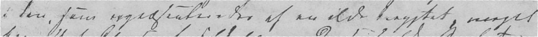i den, som repræsenteredes af en ældre berygtet, meget
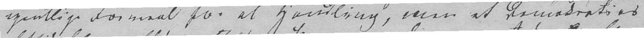egentlige Formaal for al Handling, men et Democraties
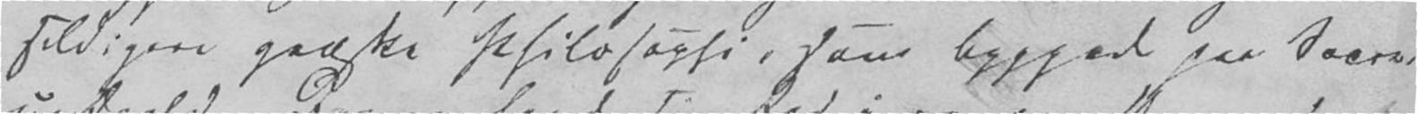sildigere græske Philosophi, som bygget paa Socra-
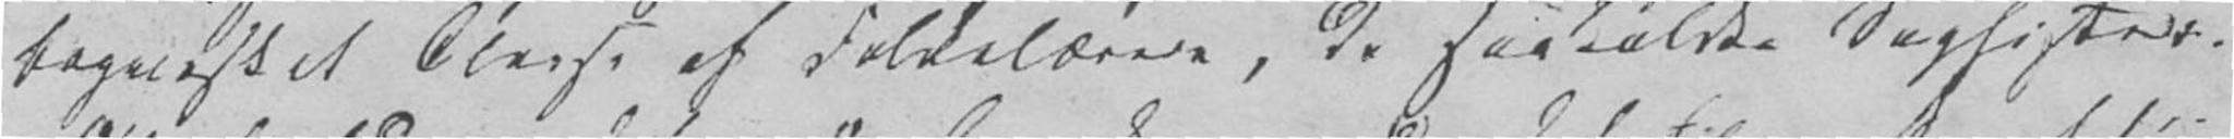bagvasket Classe af Folkelærere, de saakaldte Sophister.
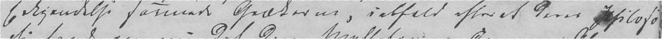Erkjendelse savnede Grækerne, ialfald efter at deres Philoso-
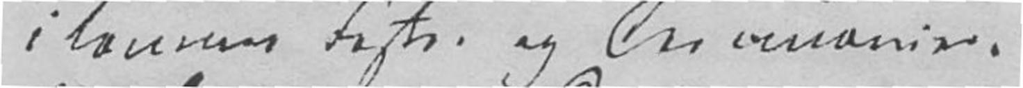i tomme Fester og Ceremonier.
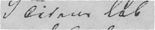I Tidens Løb
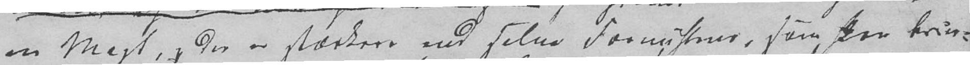en Magt, g der er stærkere end selve Fornuften, som kan brin-
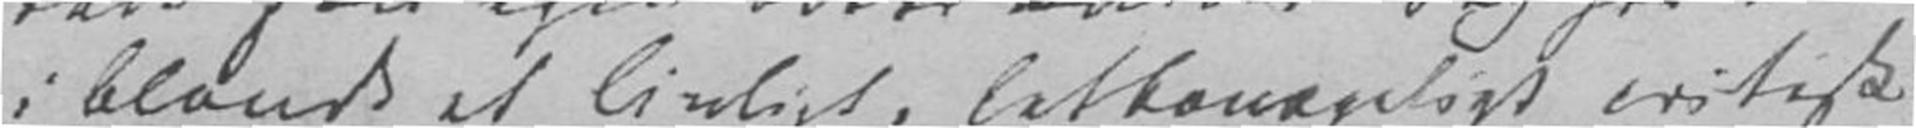i blandt et livligt, letbevægeligt critisk
